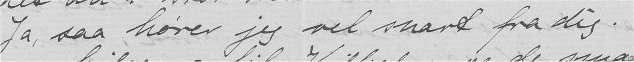Ja, saa hører jeg vel snart fra dig.
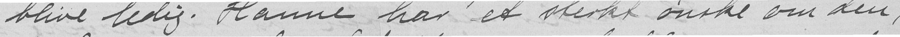blive ledig. Hanne har et sterkt ønske om den,
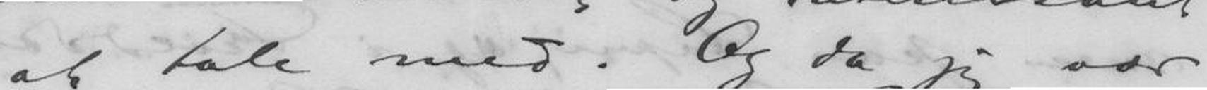at tale med. Og da jeg var
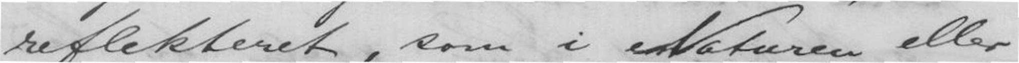reflekteret, som i Naturen eller
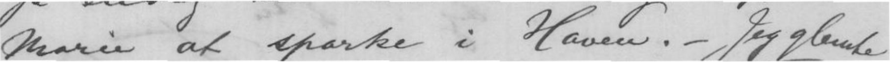Marie at sparke i Haven. - Jeg glemte
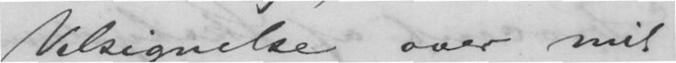Velsignelse over mit
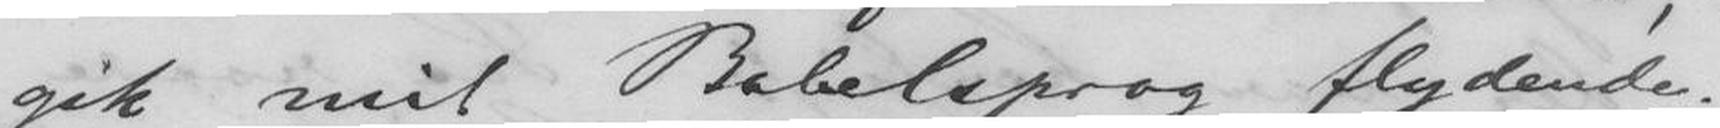gik mit Babelsprog flydende.
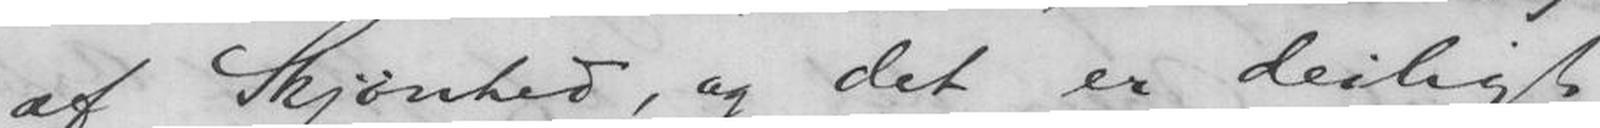af Skjønhed, og det er deiligt
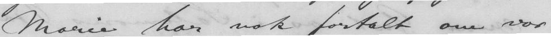Marie har nok fortalt om vor
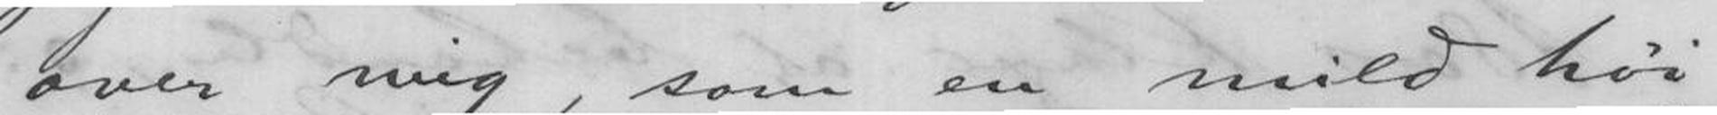over mig, som en mild høi
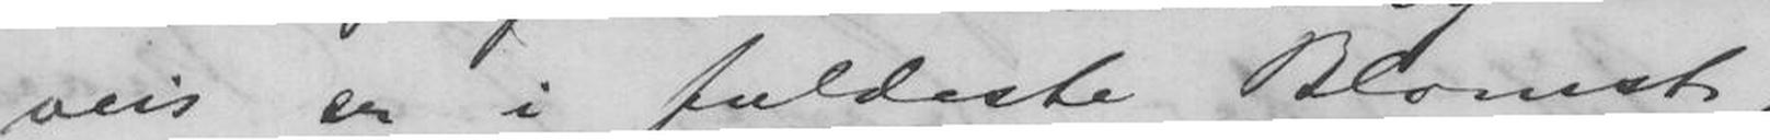veis er i fuldeste Blomst,
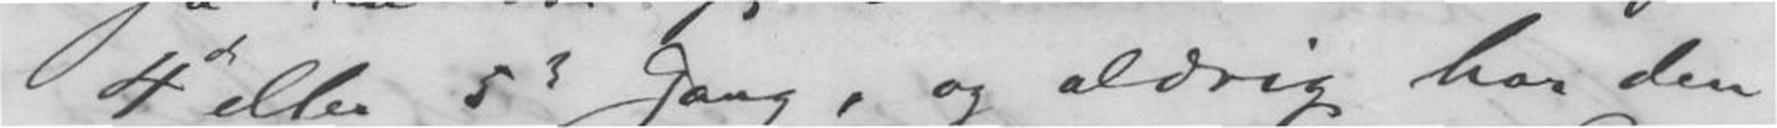4de eller 5te Gang, og aldrig har den
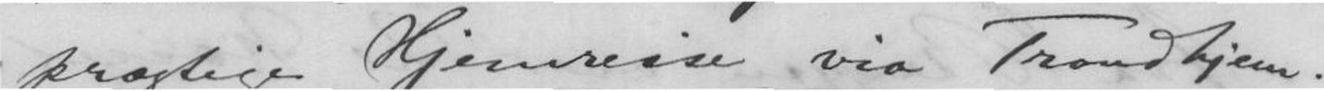prægtige Hjemreise via Trondhjem.
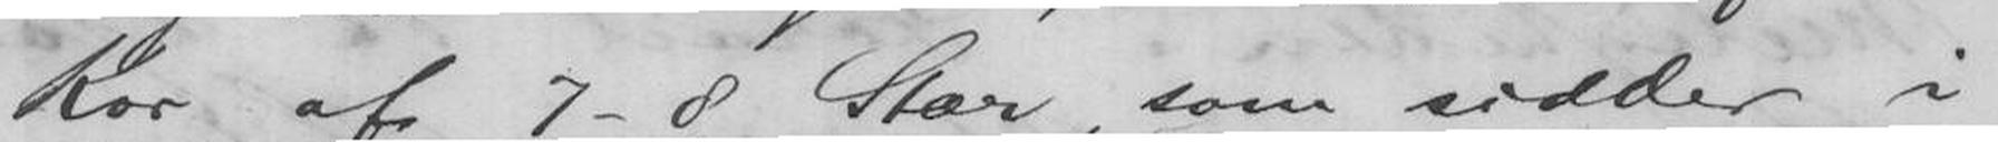Kor af 7-8 Stær, som sidder i
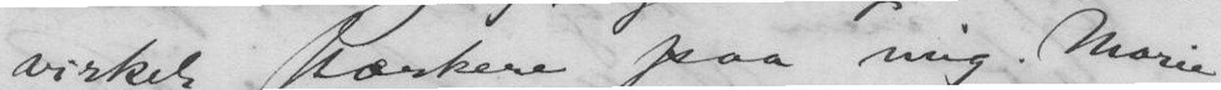virket stærkere paa mig. Marie
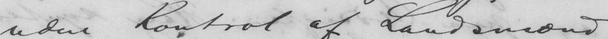uden Kontrol af Landsmænd,
